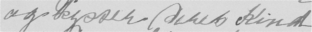og kysser deres Kind
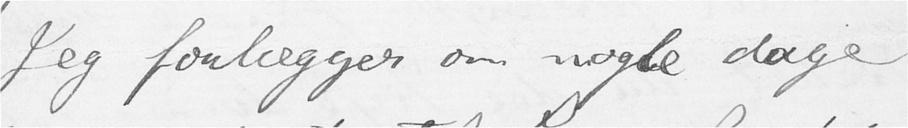Jeg forlægger om nogle dage
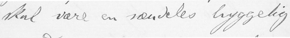skal være en særdeles hyggelig
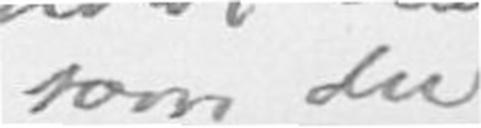som du.
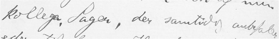kollega, Sagen, der samtidig anbefaler
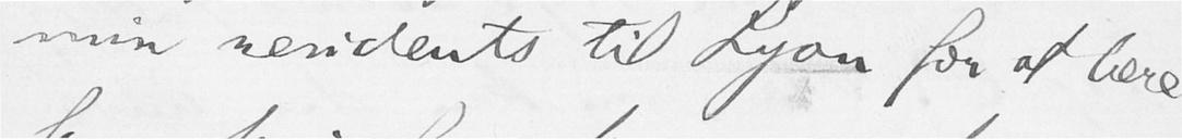min residents til Lyon for at lære
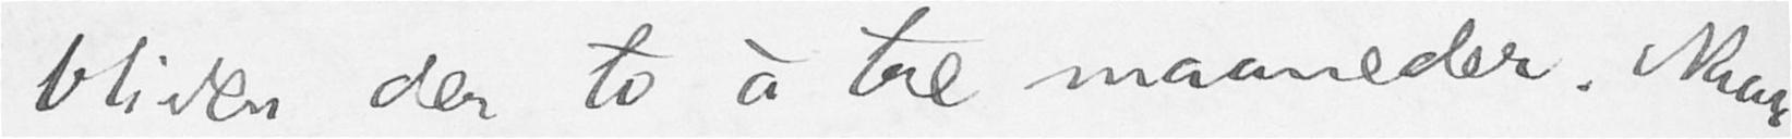bliver der to à tre maaneder. Naar
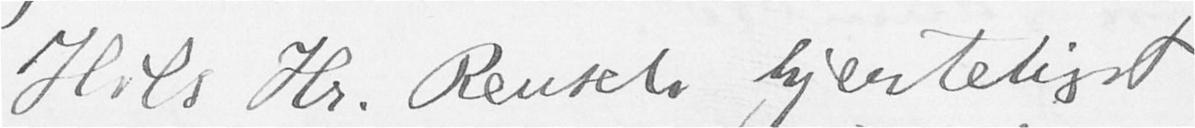Hils Hr. Reusch hjerteligst
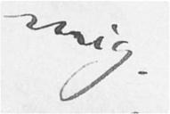mig.
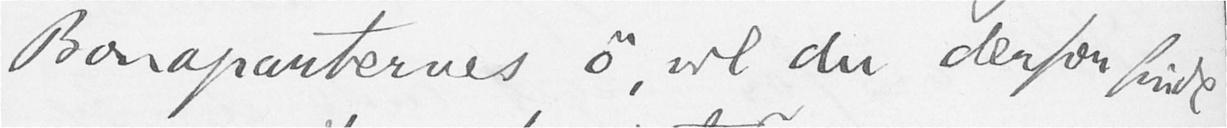Bonaparternes ø, vil du derfor finde
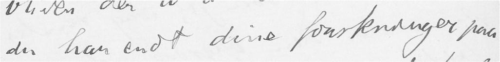du har endt dine forskninger paa
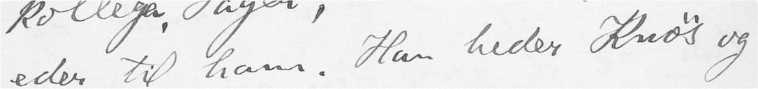eder til ham. Han heder Knøs og
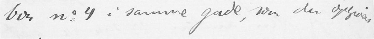bor no 4 i samme gade, som du opgiver
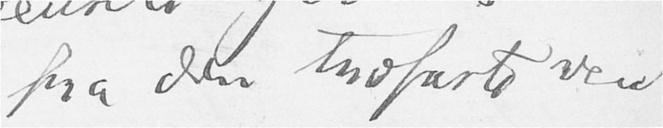fra din trofaste ven,
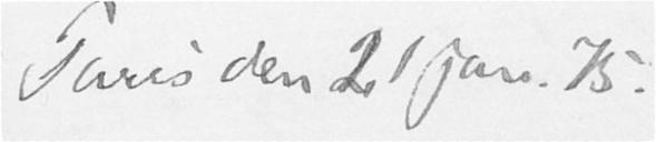Paris den 21 jan. 75.
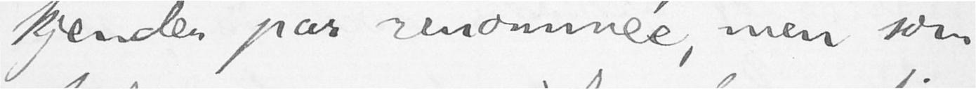kjender par renommée, men som
